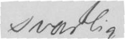svarlig
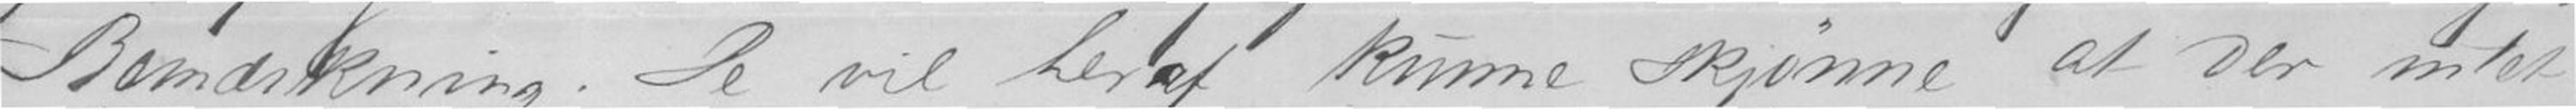Bemærkning. De vil heraf kunne skjønne at der intet
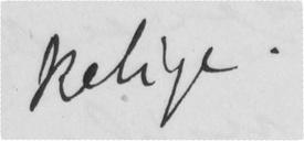kelige.
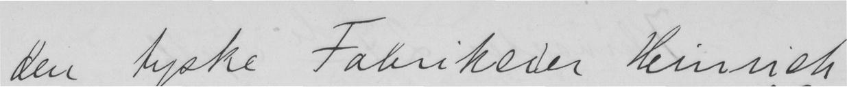den tyske Fabrikeier Heinrich
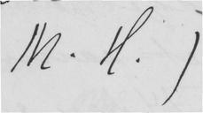M. H.)
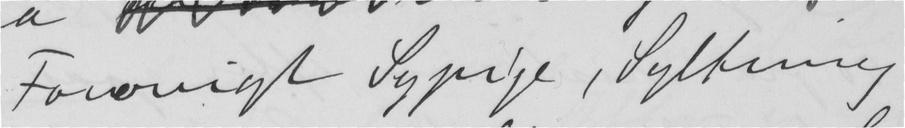Forøvrigt Sypige, Syltning
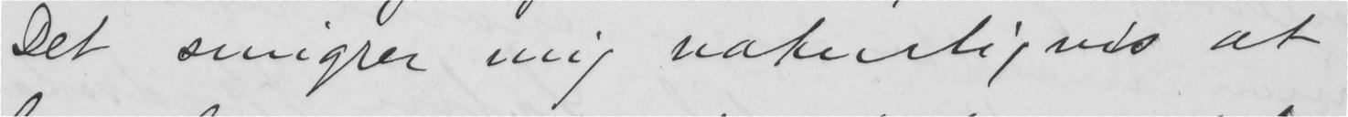Det smigrer mig naturligvis at
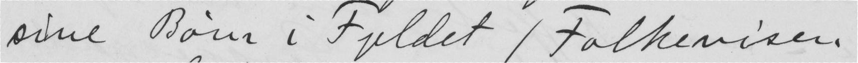sine Børn i Fjeldet (Folkeviser,
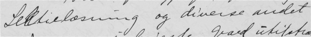Lektielæsning og diverse andet
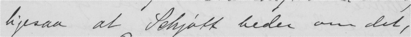ligesaa at Schjøtt beder om det,
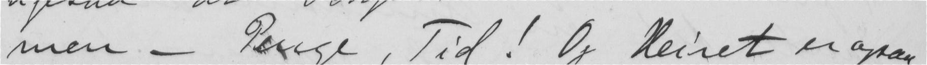men - Penge, Tid! Og Veiret er ogsaa
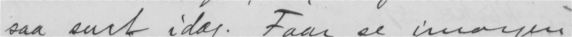saa surt idag. Faar se imorgen
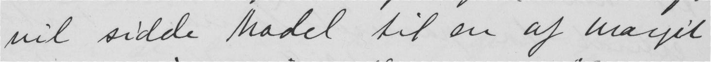vil sidde Model til en af Margit
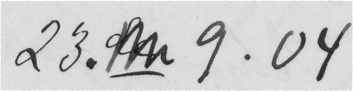23. 9.04
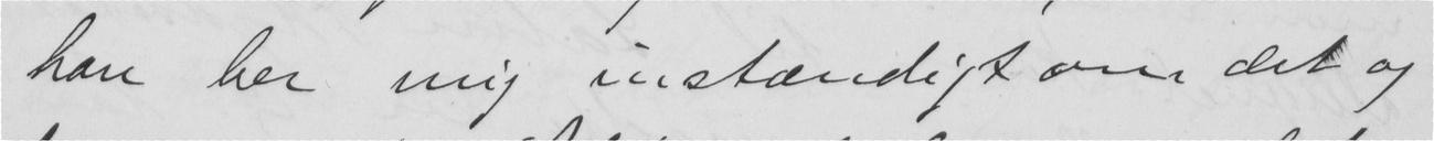han her mig instændigt om det og
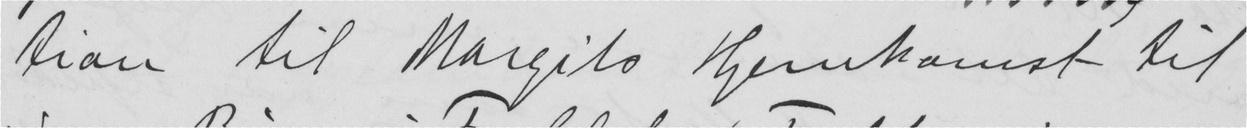tion til Margits Hjemkomst til
